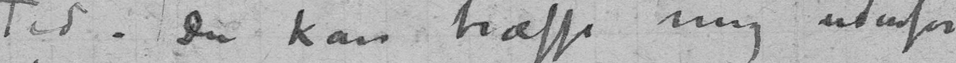Tid – Du kan træffe mig udenfor
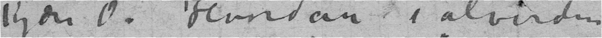Kjære 0. Hvordan i alverden
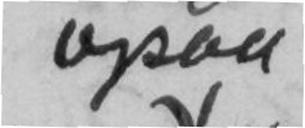ogsaa
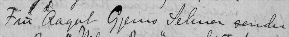Fru Aagot Gjems Selmer sender
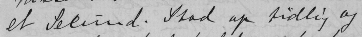et Sekund. Stod op tidlig og
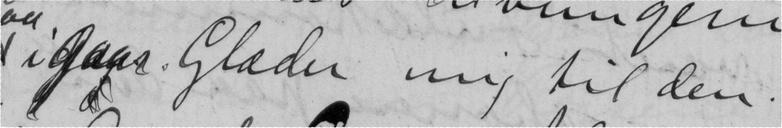i gaar. Glæder mig til den.
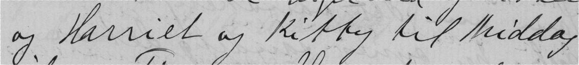og Harriet og Kitty til middag
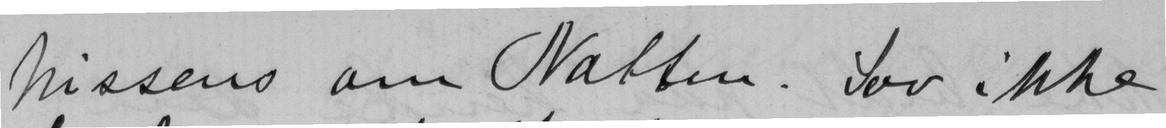Nissens om Natten. Sov ikke
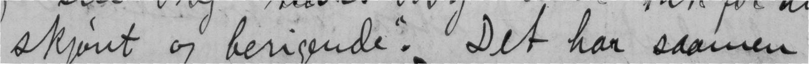skjønt og berigende". Det har saamen
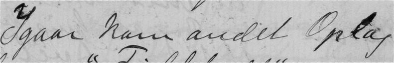Igaar kom andet oplag
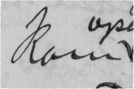kom
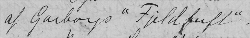af Garborgs "Fjeldluft".
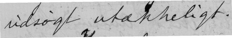udsøgt utækkeligt.
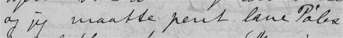og jeg maatte pent lave Pøles
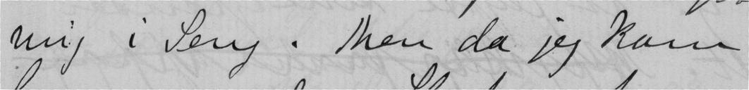mig i Seng. Men da jeg kom
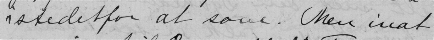i stedetfor at sove. Men inat
